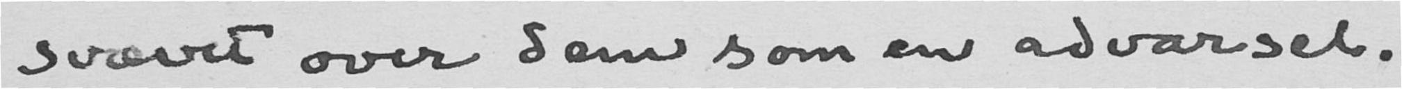svævet over dem som en advarsel.
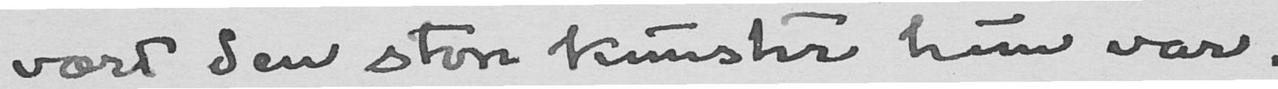vært den store kunster hun var.
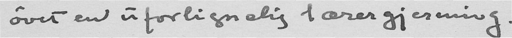øvet en uforlignelig lærergjernnng.
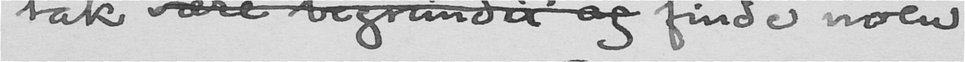tak være begrundet og finde noen
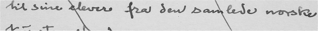til sine elever fra den samlede norske
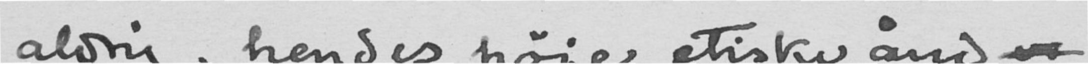aldrig, hendes høie etiske ånd
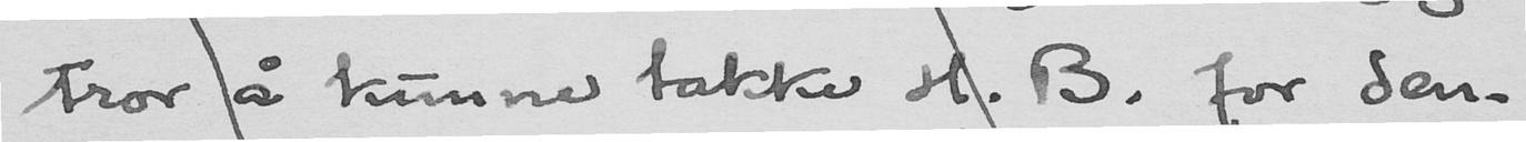tror å kunne takke H. B. for den-
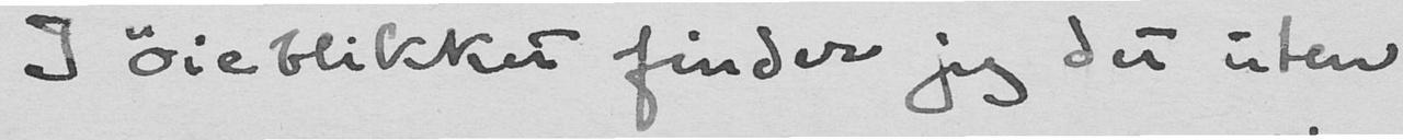I øieblikket finder jeg det uten
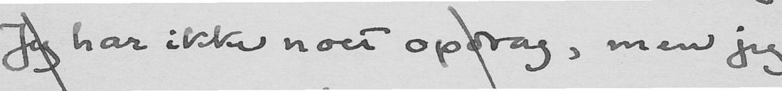Jeg har ikke noet opgrag, men jeg
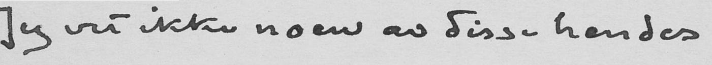Jeg vet ikke noen av disse hendes
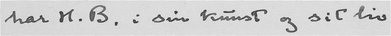har H.B. i sin kunst og sit liv
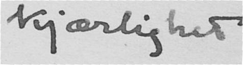kjærlighet
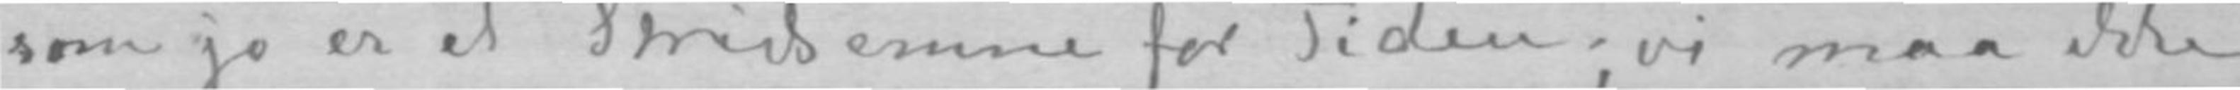som jo er et Stridsemne for Tiden; vi maa ikke
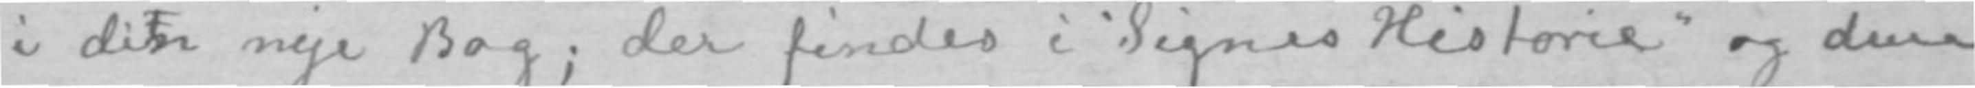i dindit nye Bog; der findes i «Signes Historie» og dine
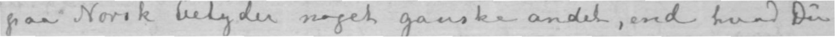paa Norsk betyder noget ganske andet, end hvad Du
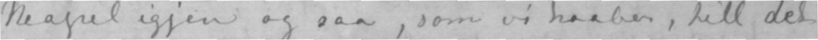Neapel igjen og saa, som vi haaber, till det
